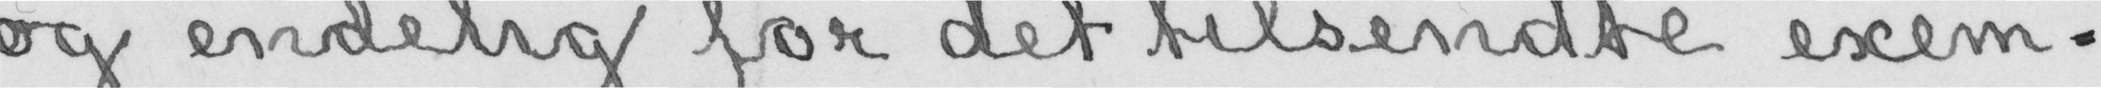og endelig for det tilsendte exem-
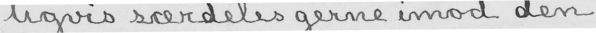ligvis særdeles gerne imod den
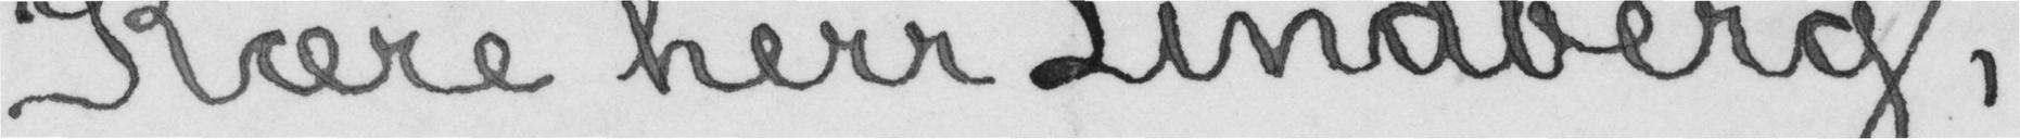Kære herr Lindberg,
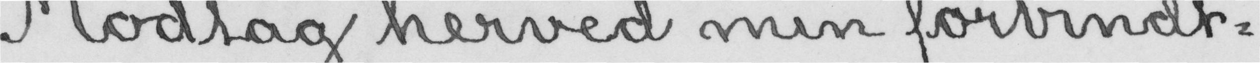Modtag herved min forbindt-
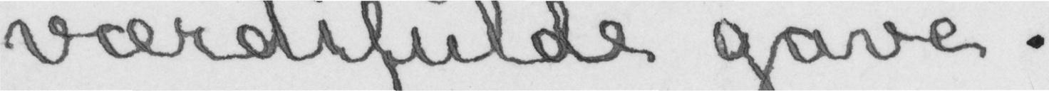værdifulde gave.
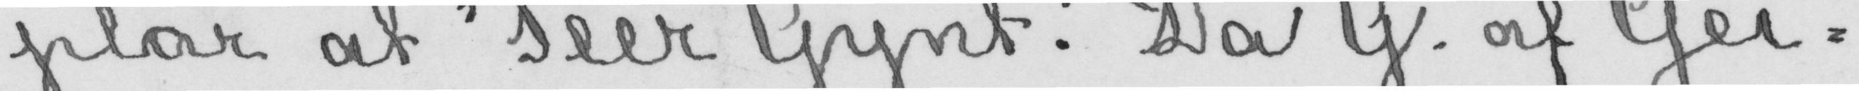plar at «Peer Gynt». Da G. af Gei-
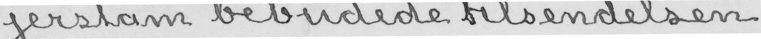jerstam bebudede tilsendelsen
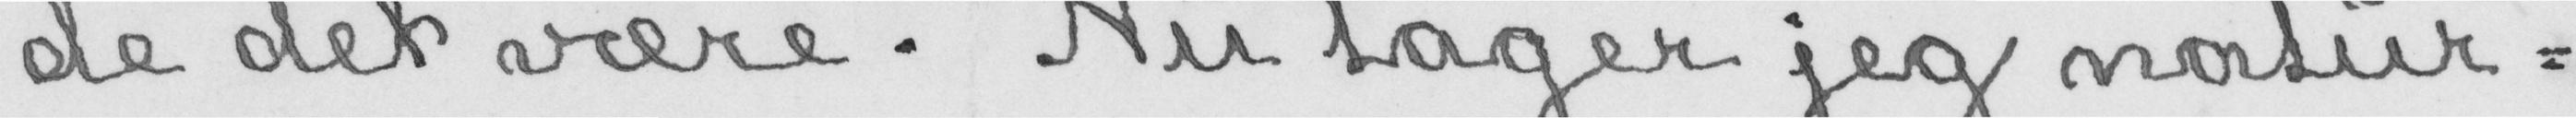de det være. Nu tager jeg natur-
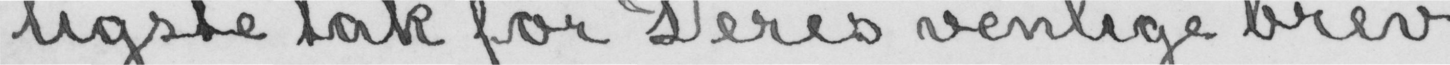ligste tak for Deres venlige brev
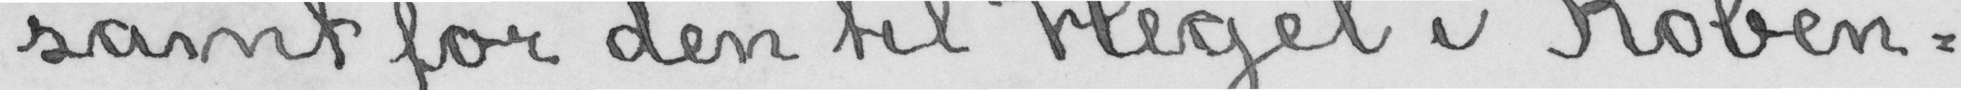samt for den til Hegel i Køben-
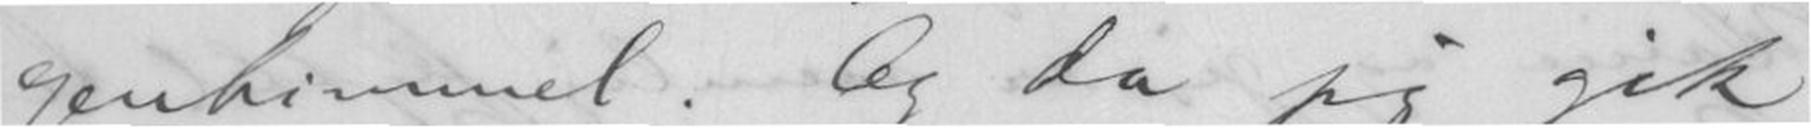genhimmel. Og da jeg gik
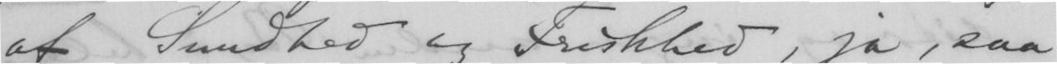af Sundhed og Friskhed, ja, saa
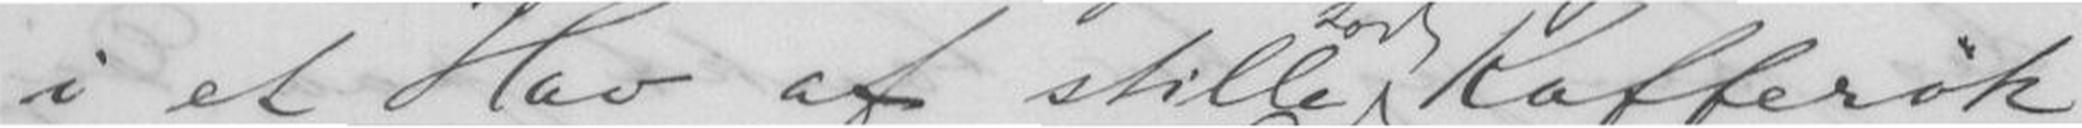i et Hav af stille Kafferøk
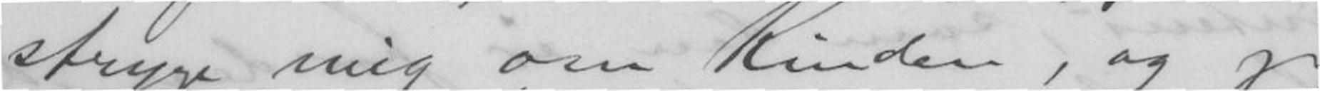stryge mig om Kinden, og jeg
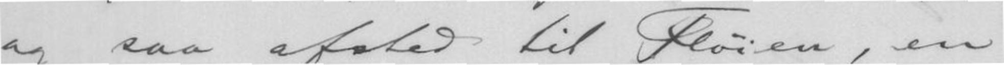og saa afsted til Fløien, en
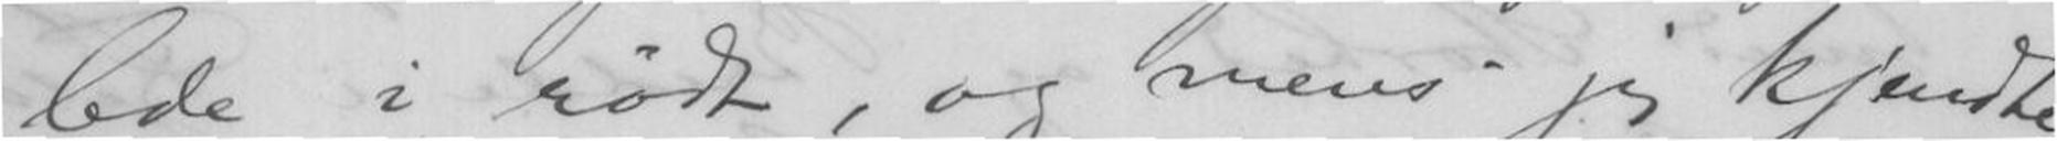lede i rødt, og mens jeg kjendte
